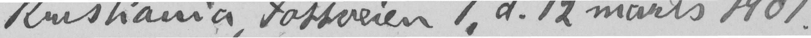Kristiania, Fossveien 7, d. 12 marts 1901
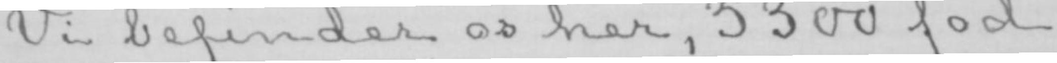Vi befinder os her, 3300 fod
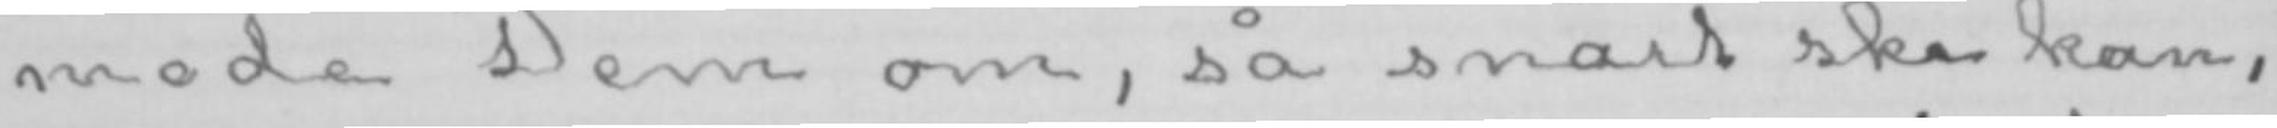mode Dem om, så snart ske kan,
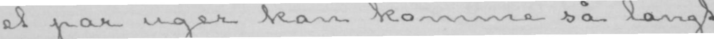et par uger kan komme så langt
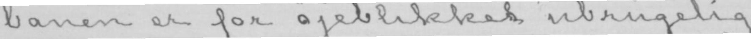banen er for øjeblikket ubrugelig
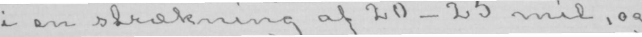i en strækning af 20-25 mil, og
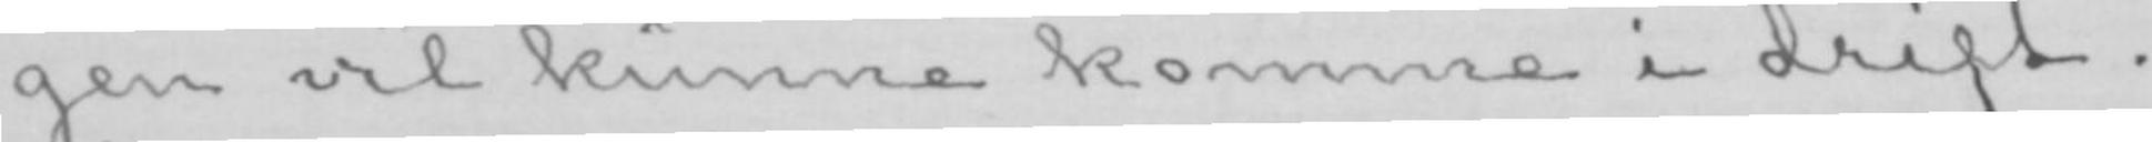gen vil kunne komme i drift.
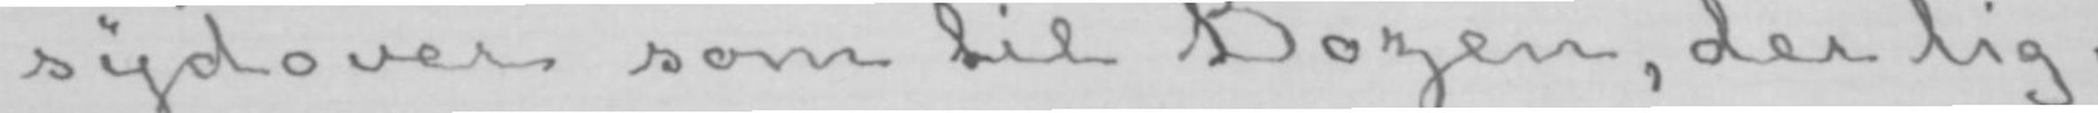sydover som til Bozen, der lig-
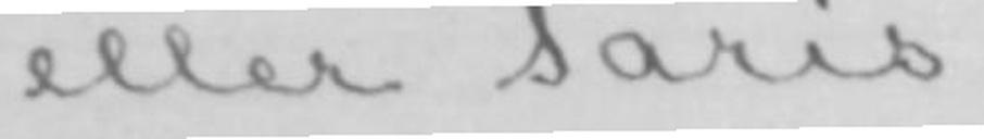eller Paris.
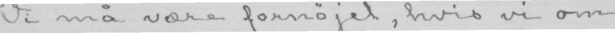Vi må være fornøjet, hvis vi om
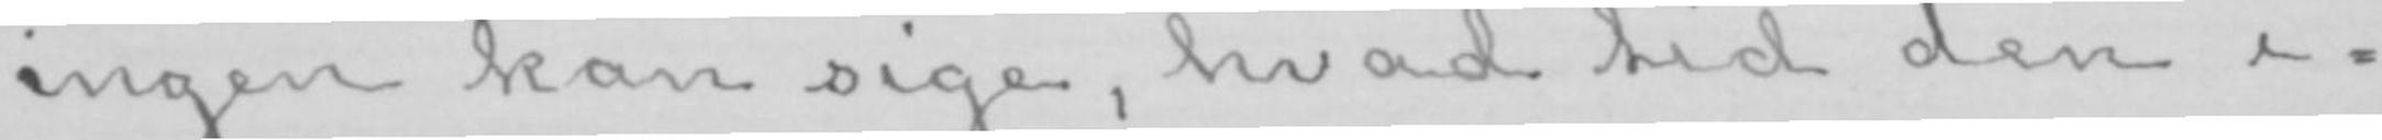ingen kan sige, hvad tid den i-
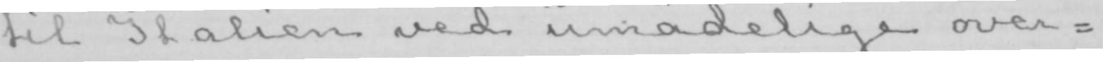til Italien ved umådelige over-
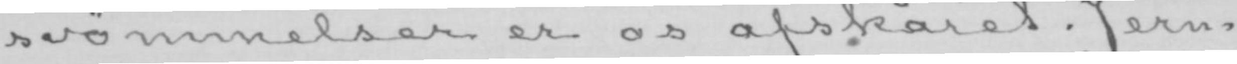svømmelser er os afskåret. Jern-
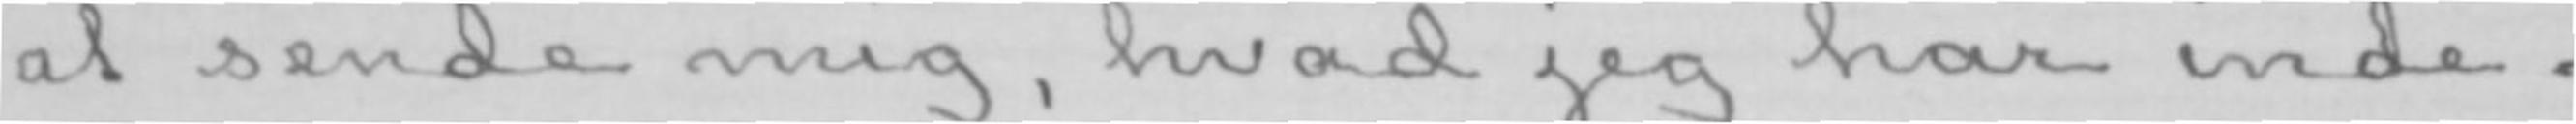at sende mig, hvad jeg har inde-
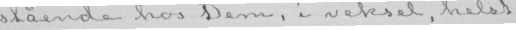stående hos Dem, i veksel, helst
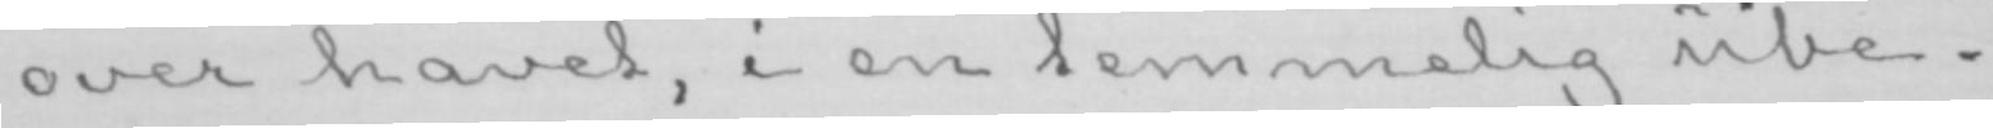over havet, i en temmelig ube-
